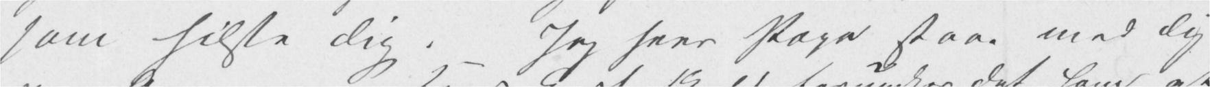som hilste Dig. Jeg seer Papa staae med Dig
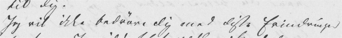Jeg vil ikke bedrøve Dig med disse Erindringer
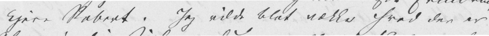kjere Robert. Jeg vilde blot vække hvad der er
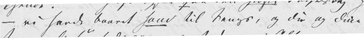– vi havde baaret ham til Sengs, og Du og Dine
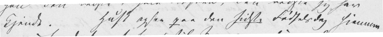kjendt. Husk ogsaa paa den sidste Fødselsdag hjemme
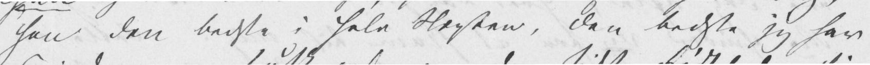han den bedste i hele Slægten, den bedste jeg har
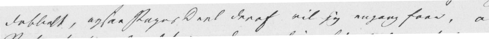dobbelt, ogsaa Papas Deel deraf vil jeg engang faae, o
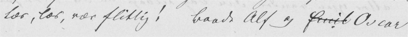Læs, læs, vær flittig! Baade Alf og Emil Oscar
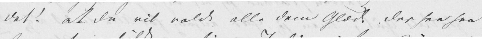det! at Du vil volde alle dem Glæde der see saa
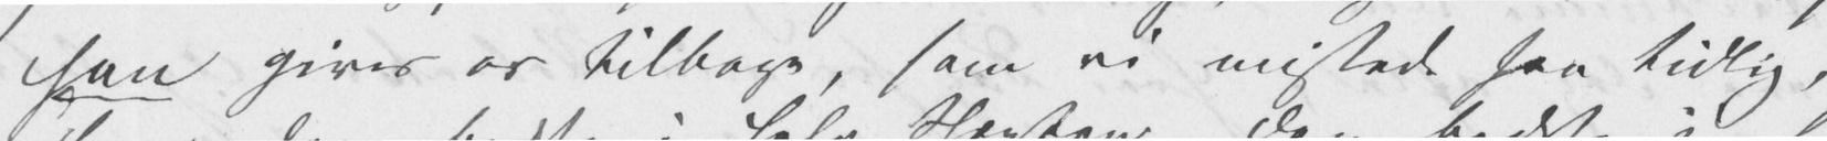han gives os tilbage, som vi mistede saa tidlig,
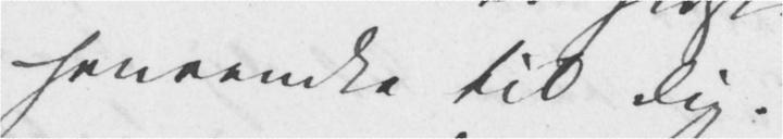henvendte til Dig.
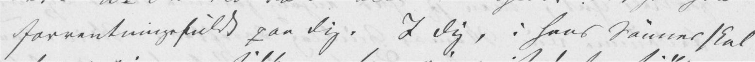forventningsfuldt paa Dig. I Dig, i hans Sønner skal
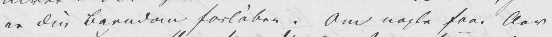er Din Barndom forløben. Om nogle faae Aar
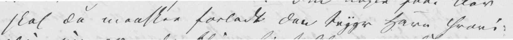skal Du maaske forlade den tryge Havn hvori
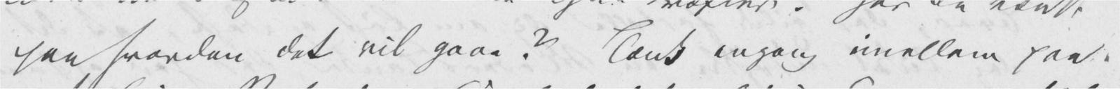paa hvordan det vil gaae? Tænk engang imellem paa
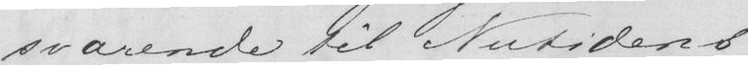svarende til Nutidens
